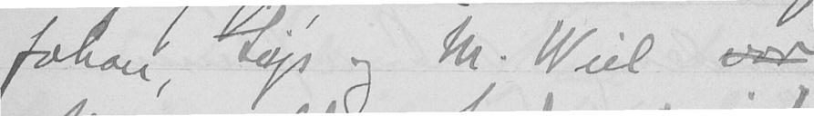Johan, Seips og M. Wiel var
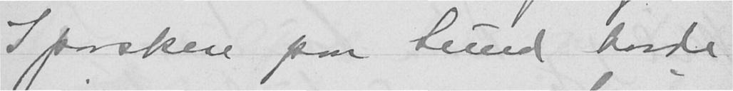I paasken paa Sund havde
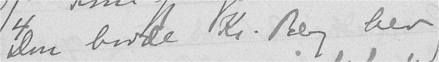Don havde Kr. Berg her
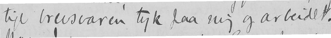tige brevsvaren tyk paa mig og arbeidet.
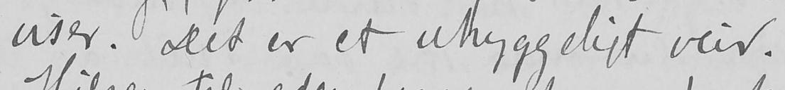viser. Det er et uhyggeligt veir.
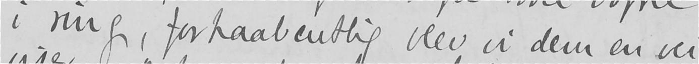i ring, forhaabentlig blev vi dem en vei-
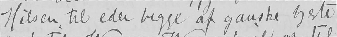Hilsen til eder begge af ganske hjerte
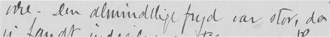være. Den almindelige fryd var stor, da
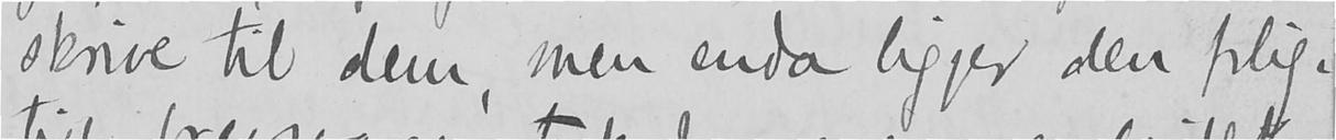skrive til dem, men enda ligger den plig-
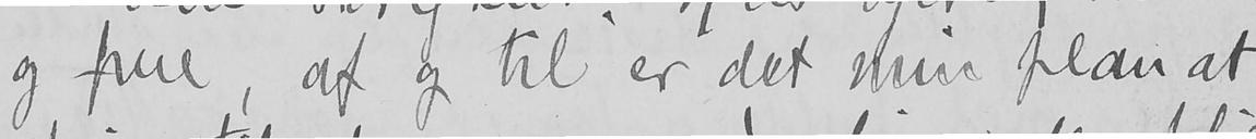og frue, af og til er det min plan at
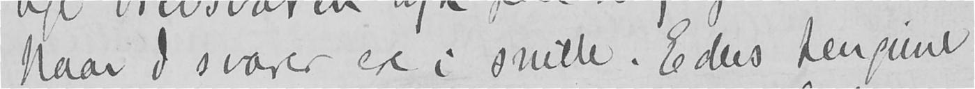Naar I svarer ere i snille. Eders hengivne
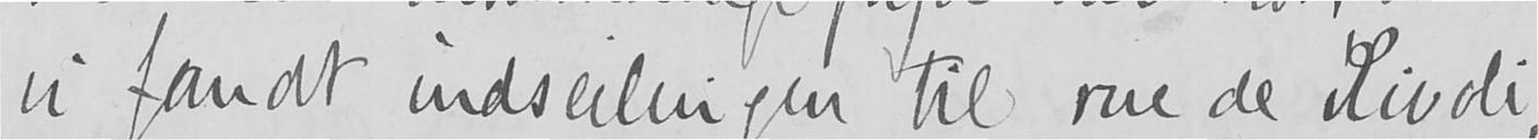vi fandt indseilingen til rue de Rivoli,
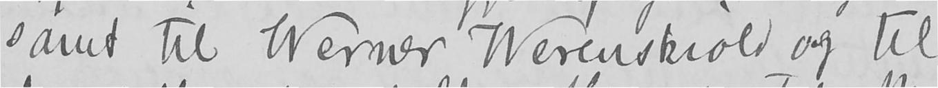samt til Werner Werenskiold og til
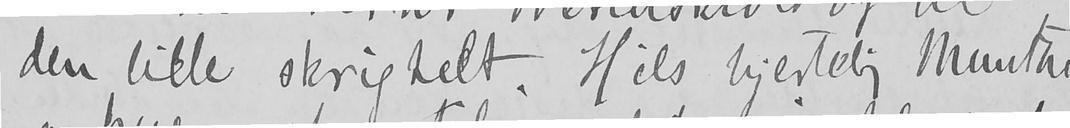den lille skrighelt. Hils hjertelig Munthe
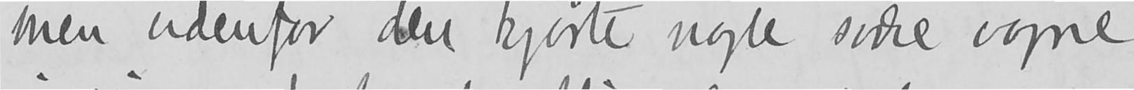men udenfor den kjørte nogle svære vogne
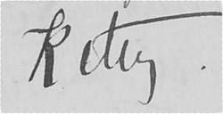Kitty.
